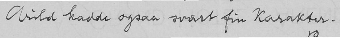Arild hadde ogsaa svært fin Karakter.
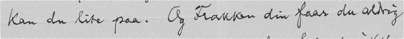kan du lite paa. Og Frakken din faar du aldrig
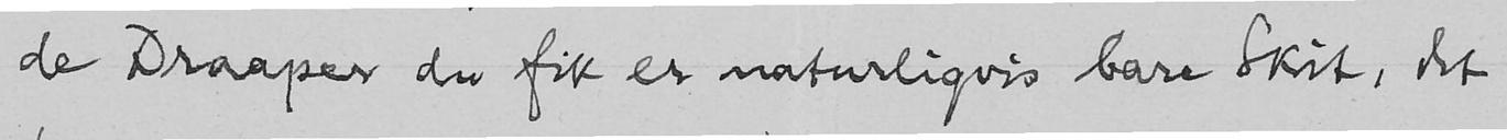de Draaper du fik er naturligvis bare Skit, det
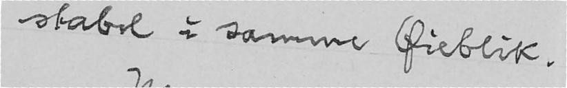stabel i samme Øieblik.
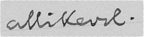allikevel.
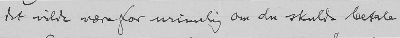det vilde være for urimelig om du skulde betale
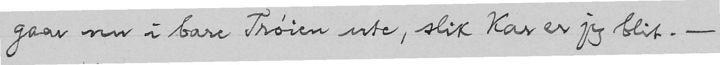gaar nu i bare Trøien ute, slik Kar er jeg blit. -
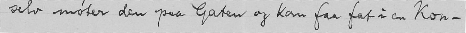selv møter den paa Gaten og kan faa fat i en Kon-
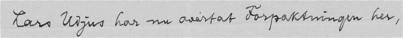Lars Udjus har nu overtat Forpaktningen her,
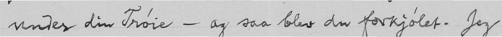under din Trøie - og saa blev du forkjølet. Jeg
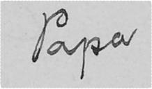Papa
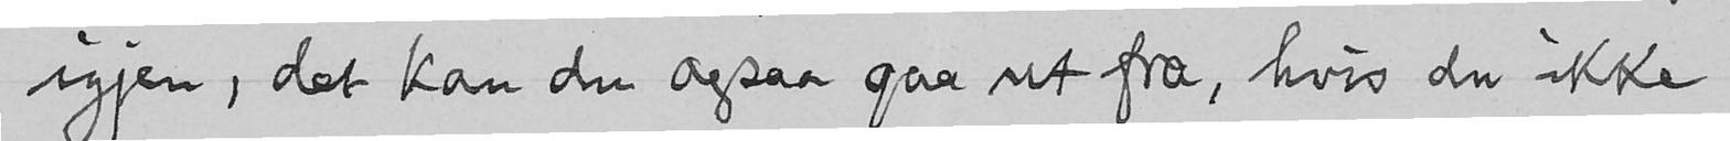igjen, det kan du ogsaa gaa ut fra, hvis du ikke
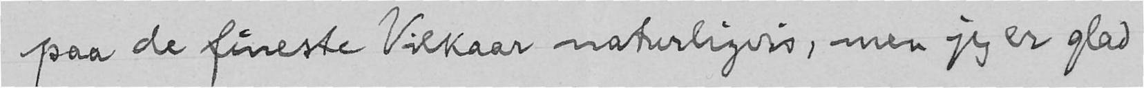paa de fineste Vilkaar naturligvis, men jeg er glad
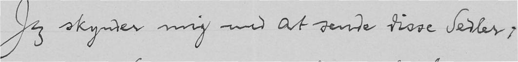Jeg skynder mig med at sende disse Sedler,
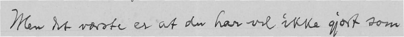Men det værste er at du har vel ikke gjort som
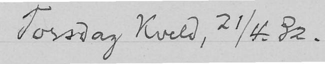Torsdag Kveld, 21/4. 32.
